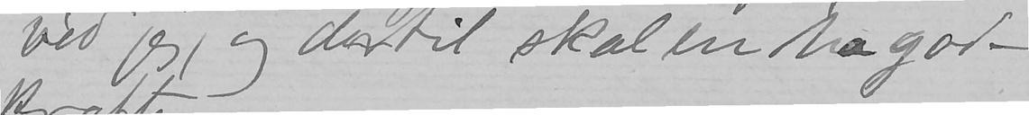véd jeg, og dertil skal en ha gode
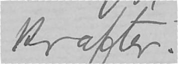kræfter.
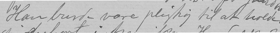Han burde være pligtig til at holde
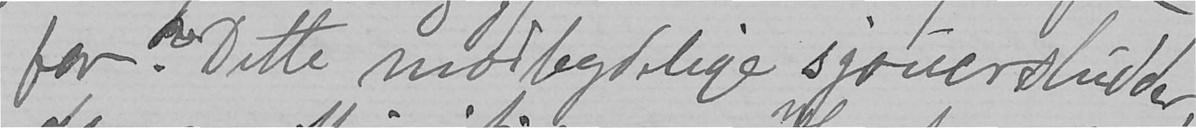for? Dette modbydelige sjouersludder,
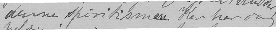denne spirtismen. Her har dog
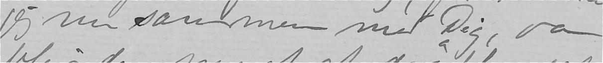jeg nu sammen med Dig, da
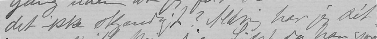det ikke skjændigt? Aldrig har jeg sét
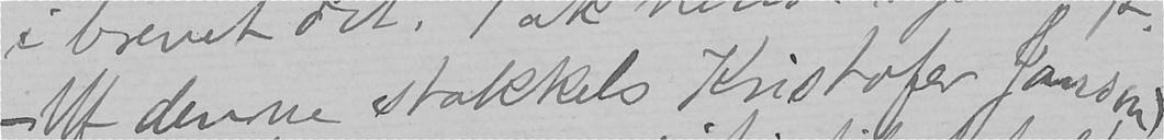- Af denne stakkels Kristofer Janson!
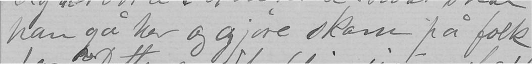han gå her og gjøre skam på folk
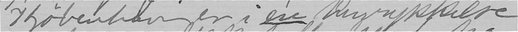Kjøbenhavn er i én henrykkelse
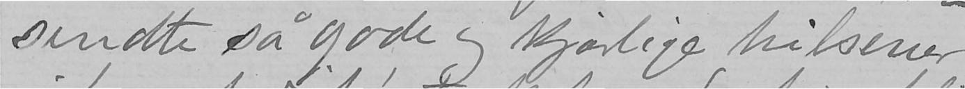sendte så gode og kjærlige hilsener
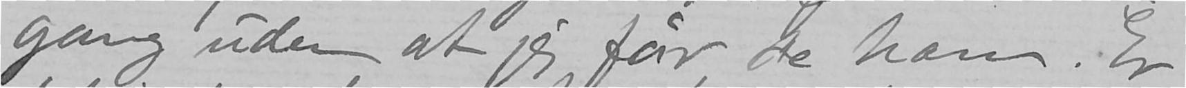gang uden at jeg får se ham. Er
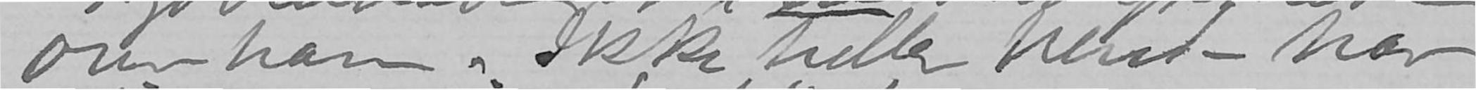over ham. Ikke heller hende har
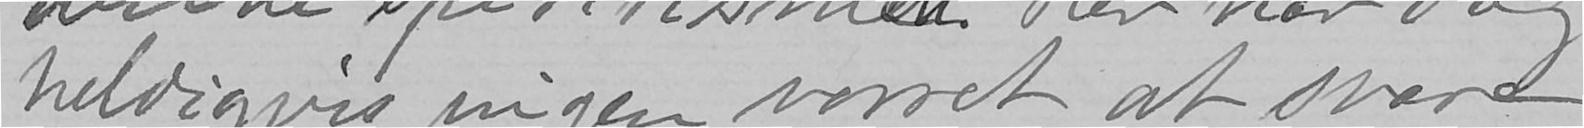heldigvis ingen vorret at svare
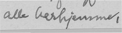alle herhjemme,
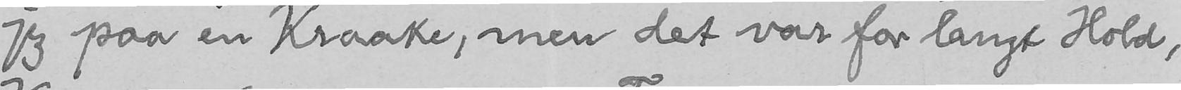jeg paa en Kraake, men det var for langt Hold,
